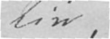Liv,
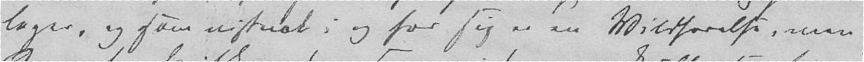loger, og som vistnok i og for sig er en Vildfarelse, men
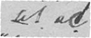at al
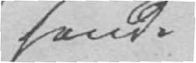havde
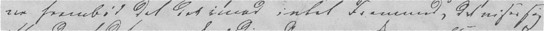ne frembød det derimod intet Fremmed, det viser sig
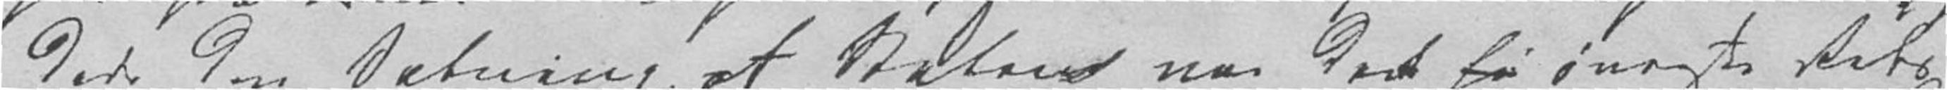dede den Sætning, at Staten var det høi øverste Rets-
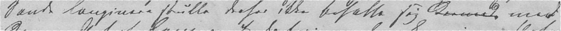Sande Lovgivere skulle derfor ikke befatte sig med
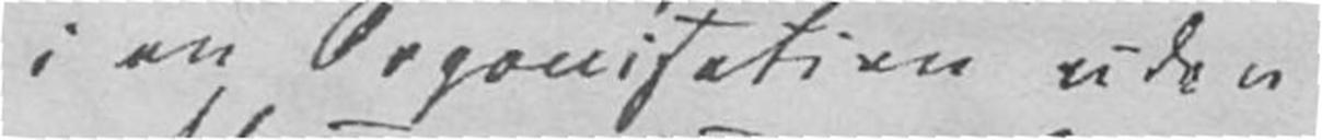i en Organisation
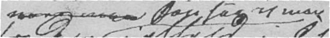Consee men
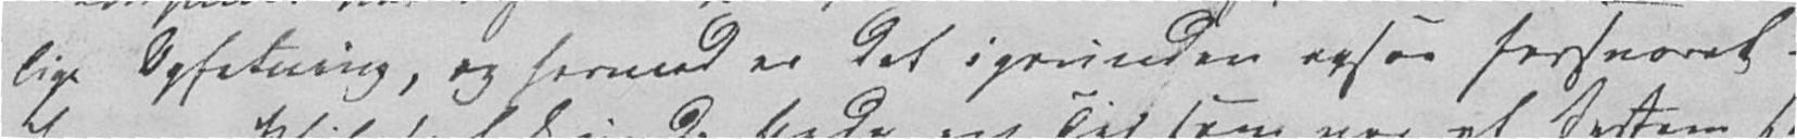lige Opfatning, og hermed er det igrunden ogsaa forsvaret.
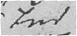Ind
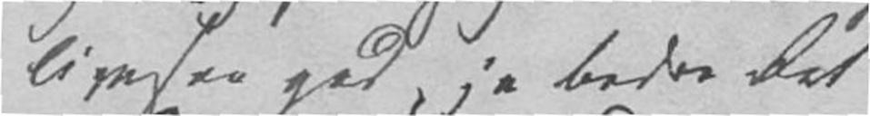ligesaa god, ja bedre Ret
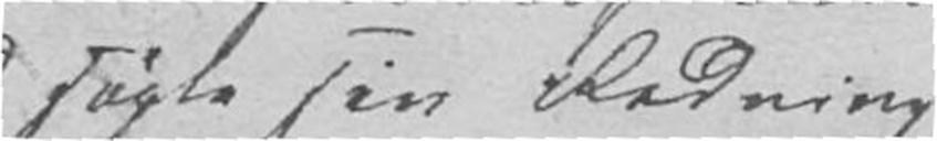søgte sin Redning
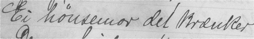Ei hønsemor det krænker
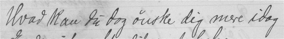Hvad kan du dog ønske dig mere idag
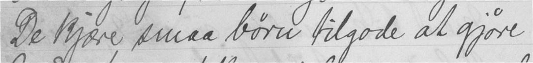De kjære smaa børn tilgode at gjøre
